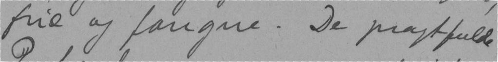frie og fangne. De pragtfulde
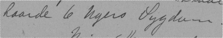haarde 6 Ugers sygdom.
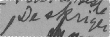De skriger
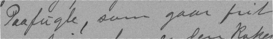Paafugle, som gaar frit
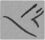12
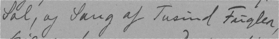Sol, og Sang af Tusind Fugler,
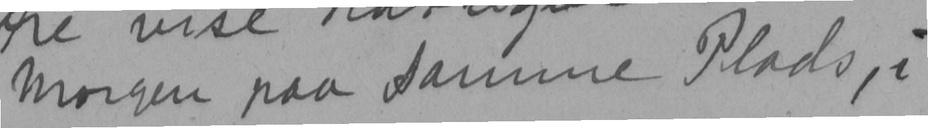morgen paa Samme Plads, i
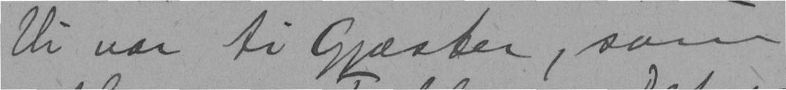Vi var ti Gjæster, som
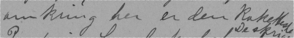omkring her er den Koketteske
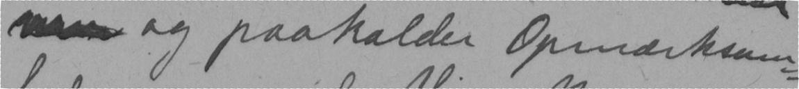og paakalder Opmærksom-
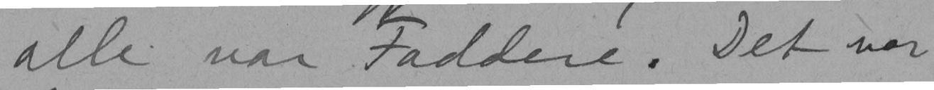alle var Faddere. Det var
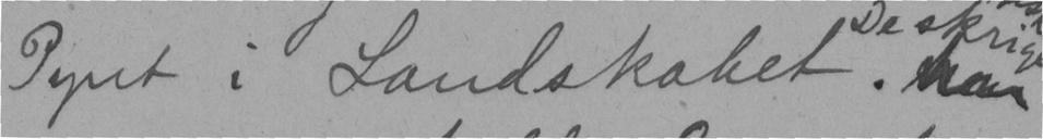Pynt i Landskabet.
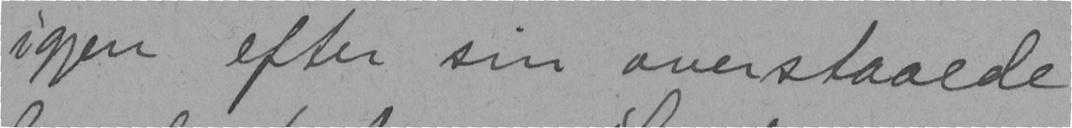igjen efter sin overstaaede
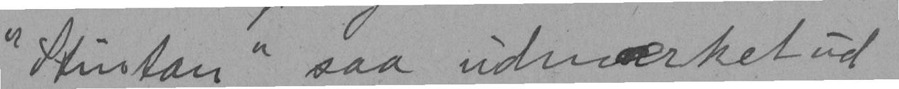"Stintan" saa udmærket ud
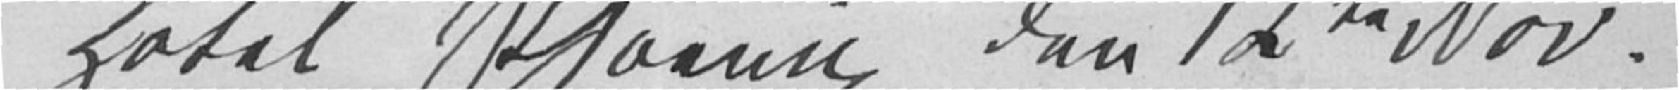Hotel Phøenix den 12te Nov.
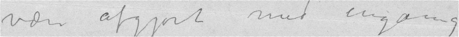være afgjort med engang
